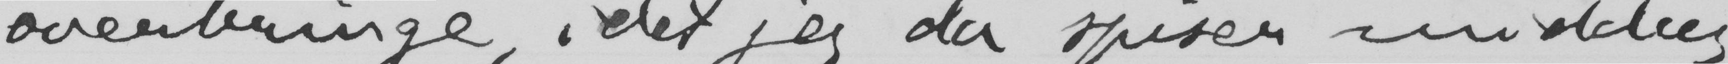overbringe, idet jeg da spiser middag
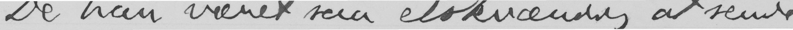De har været saa elskværdig at sende
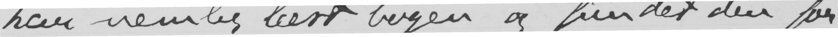har nemlig læst bogen og fundet den for-
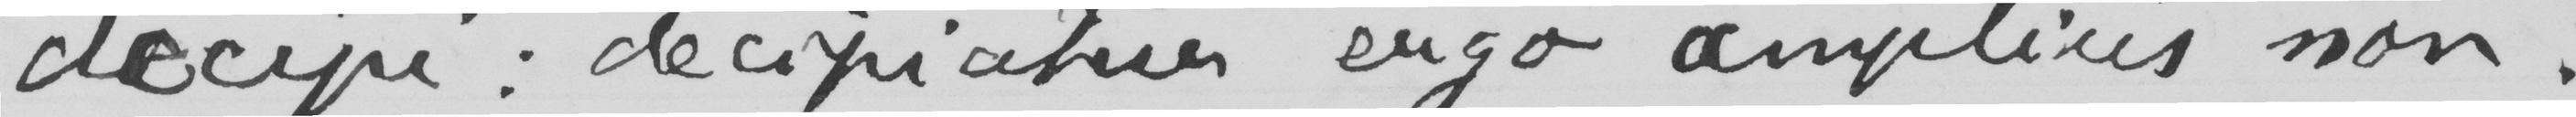decipi: decipiatur ergo amplius non!
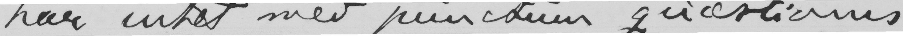har intet med punctum quæstionis
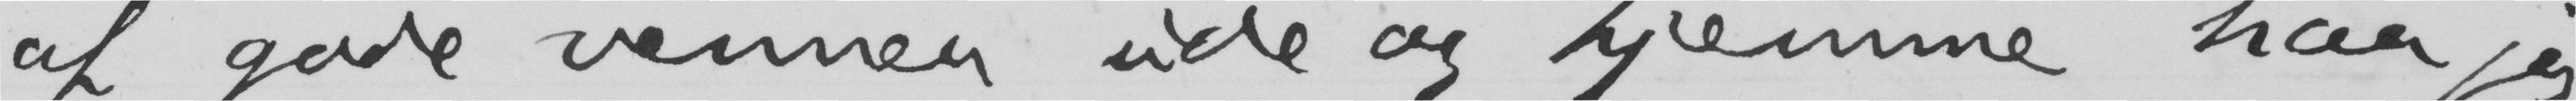af gode venner ude og hjemme har jeg
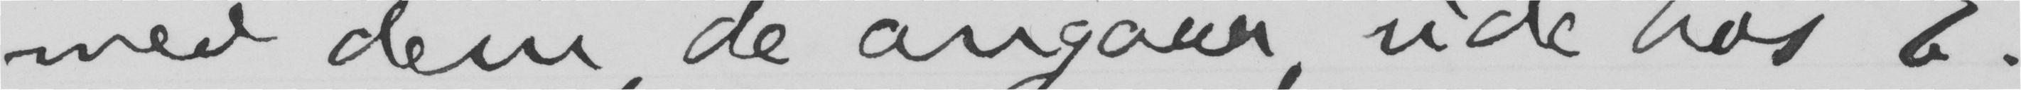med dem, de angaar, ude hos E.
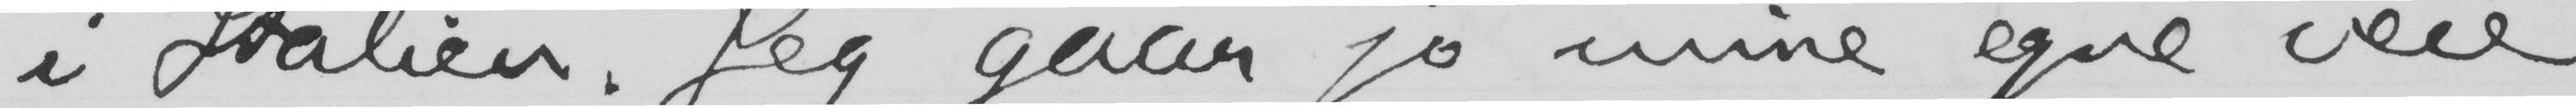i Italien. Jeg gaar jo mine egne veie,
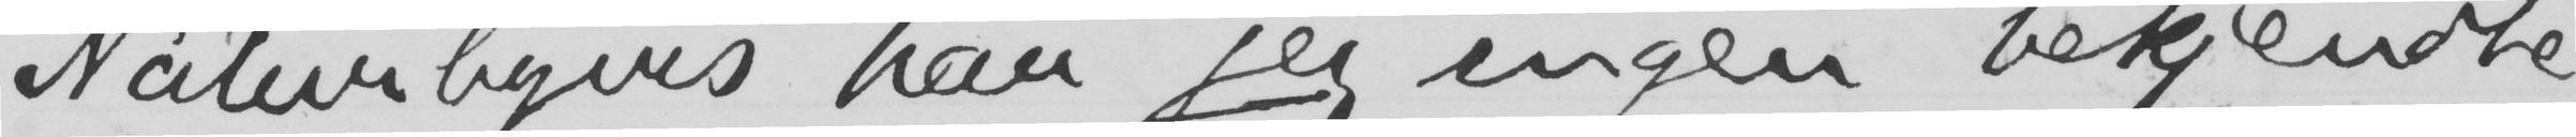Naturligvis har jeg ingen bekjendte
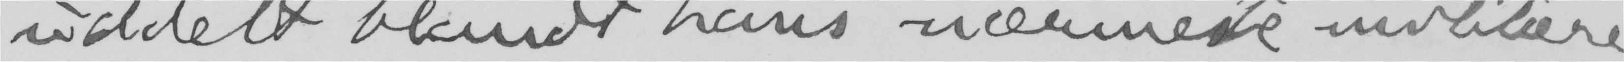uddelt blant hans nærmeste militære
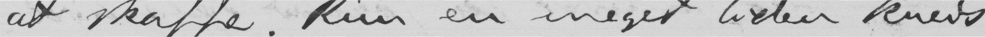at skaffe. Kun en meget liden kreds
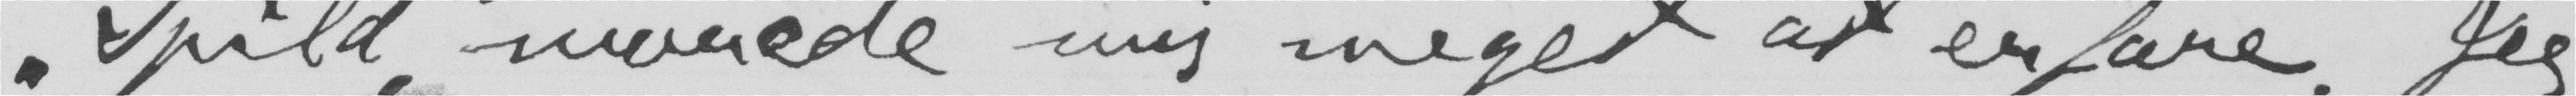«Spild» morede mig meget at erfare. Jeg
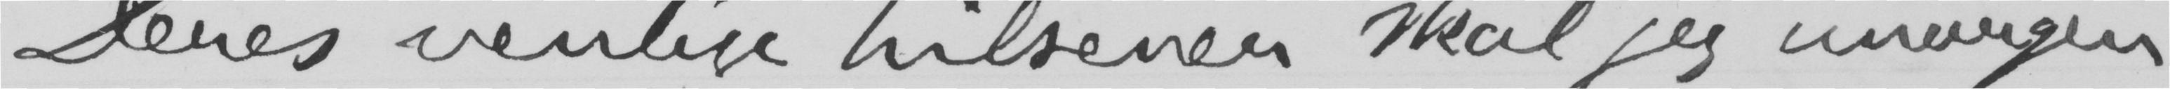Deres venlige hilsener skal jeg imorgen
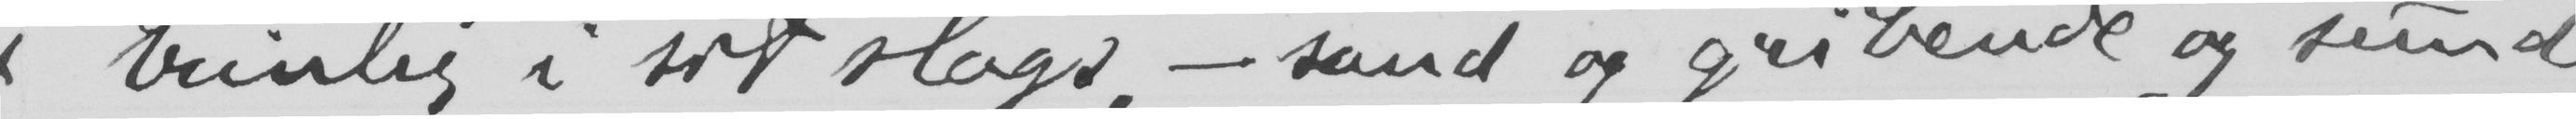trinlig i sit slags, - sand og gribende og sund
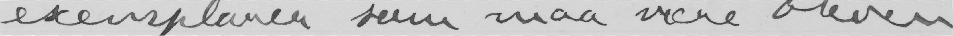exemplarer, som maa være bleven
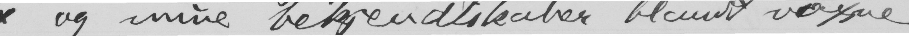og mine bekjendtskaber blandt voxne
